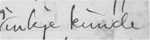inkje kunde
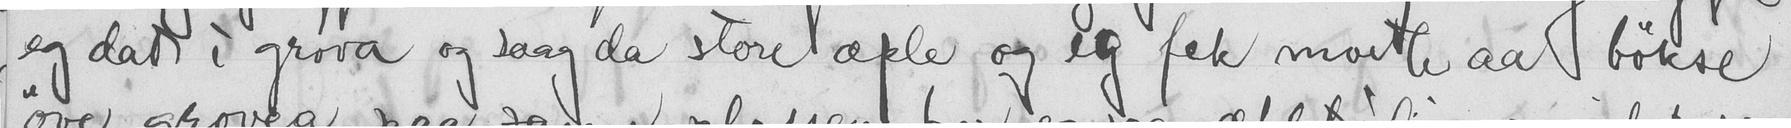og dat i grova og saag da store æple, og eg fek morte aa bøkse
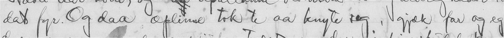dat fyr. Og daa æplinne tok te aa knyte seg, gjæk far og eg
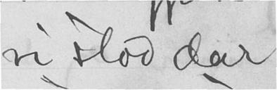vi stod dar
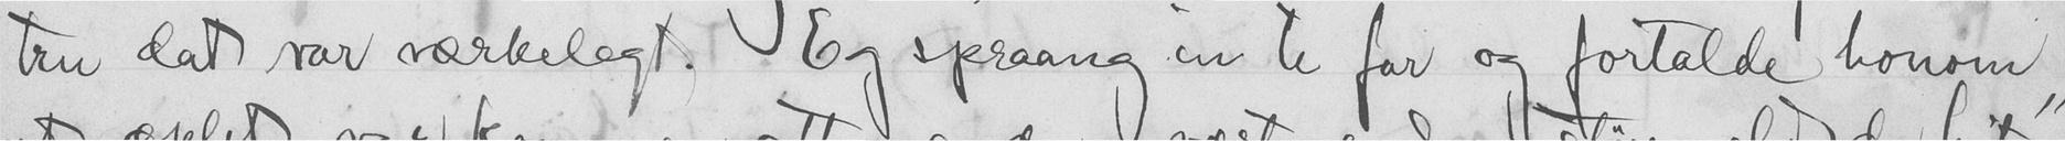tru dat var værkelegt. Eg spraag in te far og fortalde honom
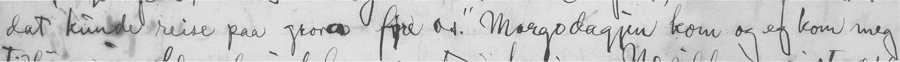dat kunde reise paa grova fyre os". Morgodagjen kom og eg kom meg
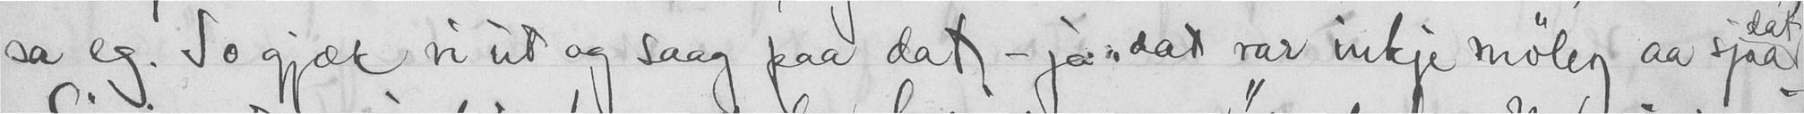sa eg. So gjæk vi ut og saag paa dat - ja, dat var inkje møleg aa sjaa
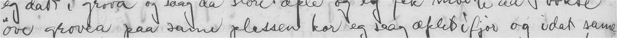øve grovea paa same plessen kor eg saag æplet ifjor og idet same
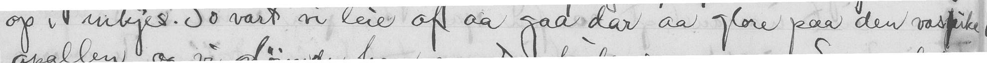op i inkjes. So vart vi leie af aa gaa dar aa glore paa den vaskike
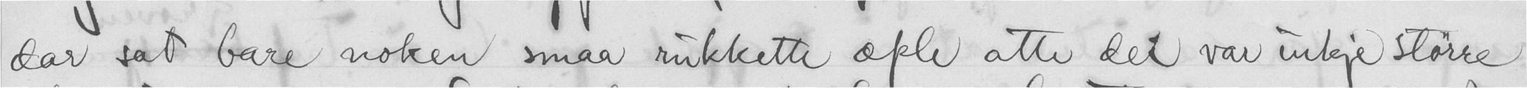dar sat bare noken smaa rukkette æple atte dei var inkje større
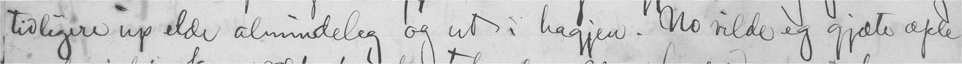tidligere op elde almindeleg og ut i hagjen. No vilde og gjæte æple
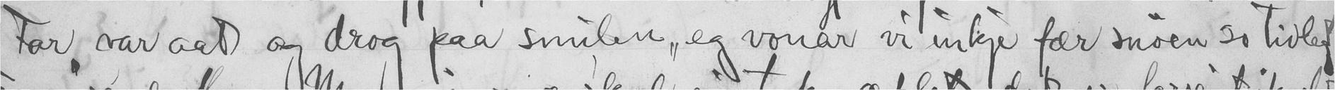Far, var aat og drog paa smilen "eg vonar vi inkje fær snøen so tidleg
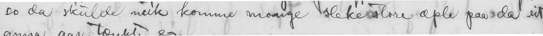so da skulde nuk komme monge sleke store æple paa da eit
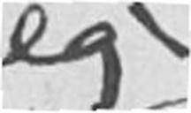eg
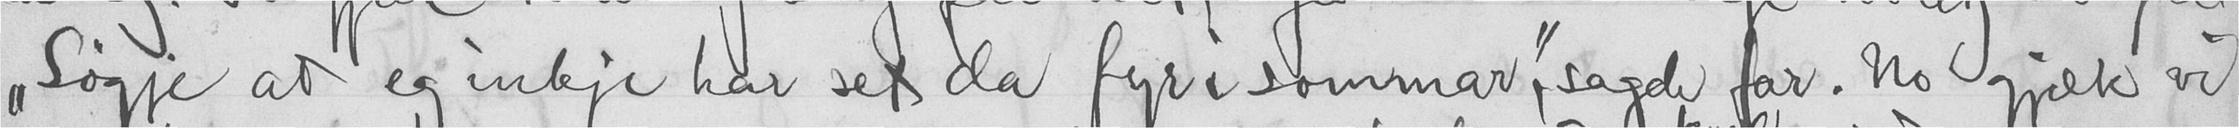"Søgje at eg inkje har set da fyr i sommar", sagde far. No gjæk vi
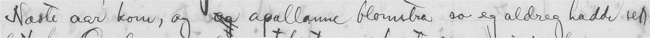Naste aar kom, og  apallanne blomstra so eg aldreg hadde set
